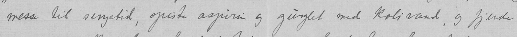messe til sengetid, spiste aspirin og gurglet med kolivand, og fjerde
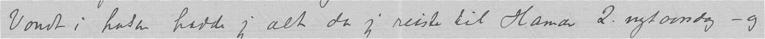Vondt i halsen hadde jeg alt da jeg reiste til Hamar 2. nytaarsdag – og
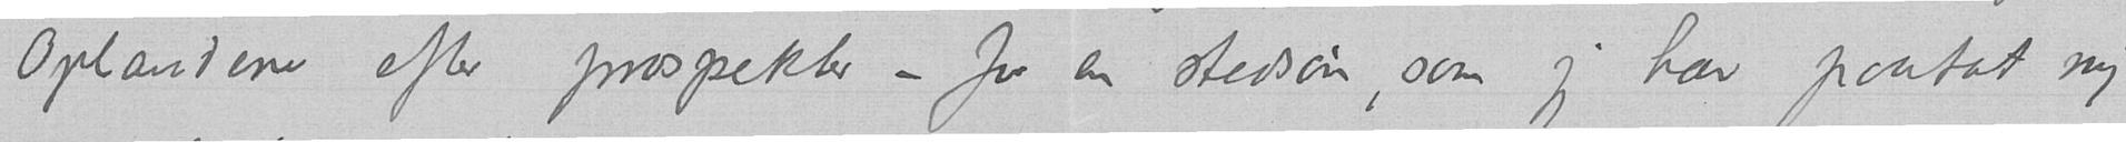Oplandene efter prospekter – for en stedsøn, som jeg har paatat mig
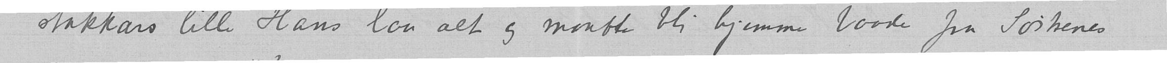stakkars lille Hans laa alt og maatte bli hjemme baade fra Søstrenes
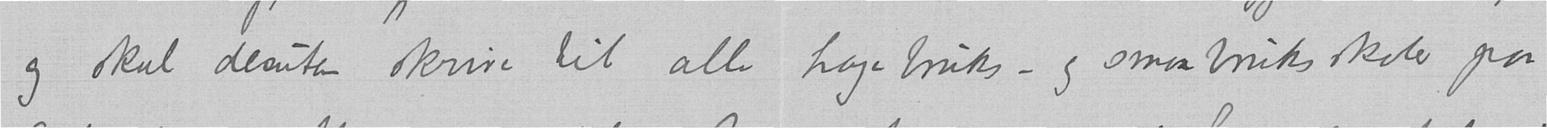og skal desuten skrive til alle hagebruks - og smaabruksskoler paa
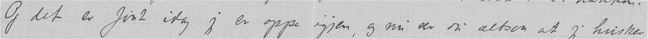Og det er først idag jeg er oppe igjen, og nu ser du altsaa at jeg husker
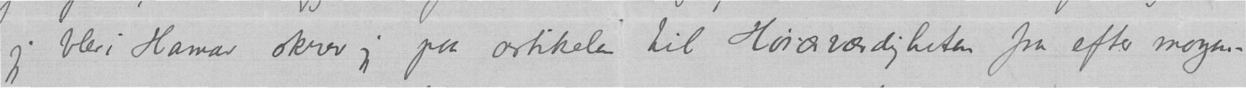jeg blev i Hamar skrev jeg paa artikelen til Høiærværdigheten fra efter morgen-
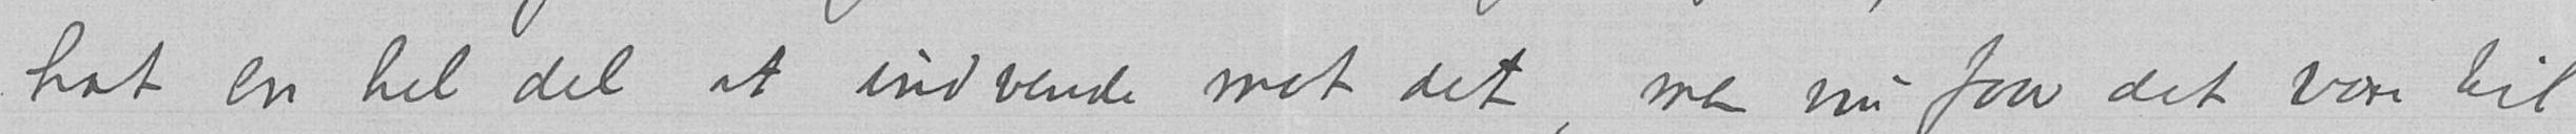hat en hel del at indvende mot det, men nu faar det være til
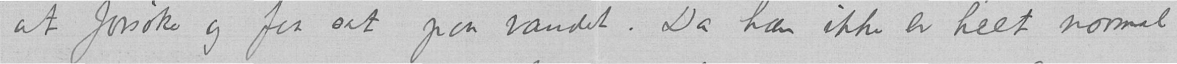at forsøke og faa sat paa vandet. Da han ikke er helt normal
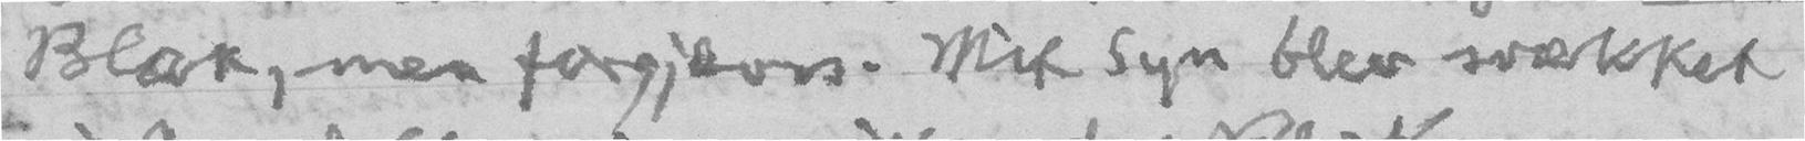Blæk, men forgjæves. Mit Syn blev svækket
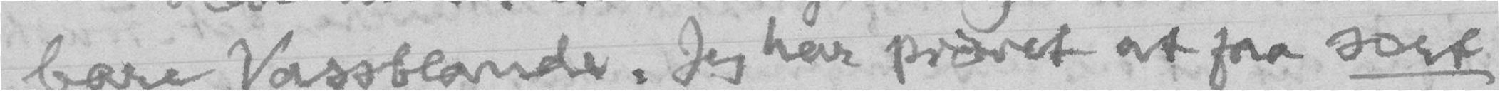bare Vassblande. Jeg har prøvet at faa sort
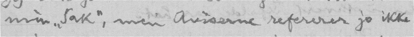min "Sak", men Aviserne refererer jo ikke
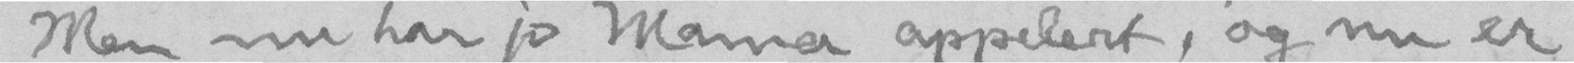Men nu har jo Mama appelert, og nu er
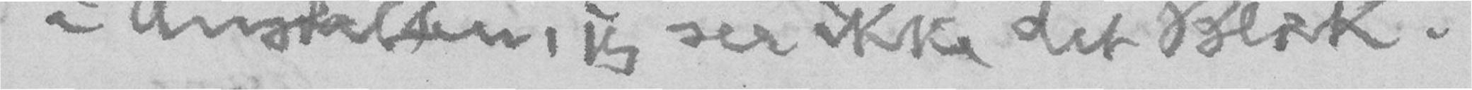i Anstalten, jeg ser ikke det Blæk.
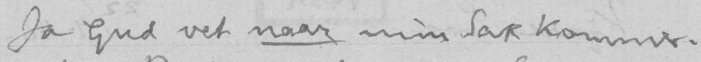Ja Gud vet naar min Sak kommer.
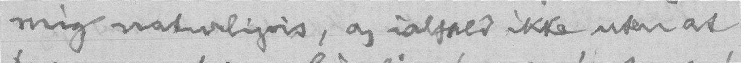mig naturligvis, og ialfald ikke uten at
